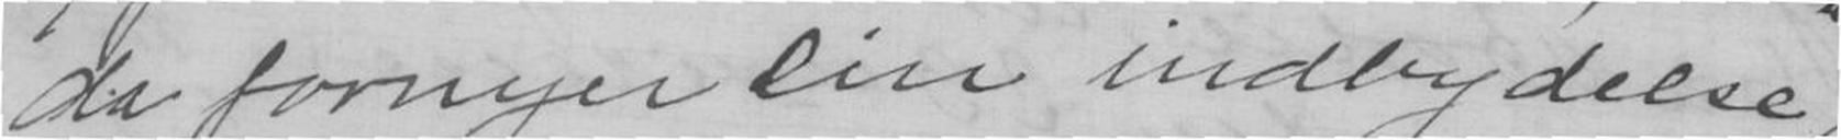da fornyer din indbydelse,
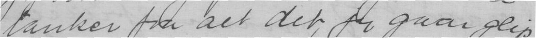tanker fra alt det, jeg gaar glip
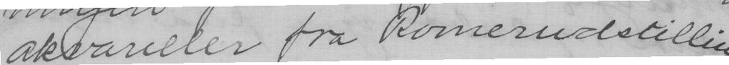akvareller fra Romerudstillin
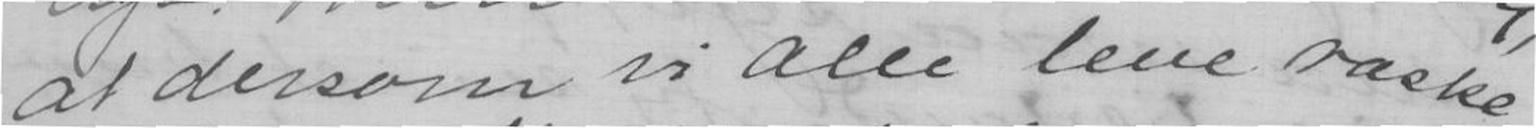at dersom vi alle leve raske
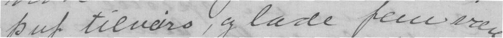puf tilveirs, og lade fem være
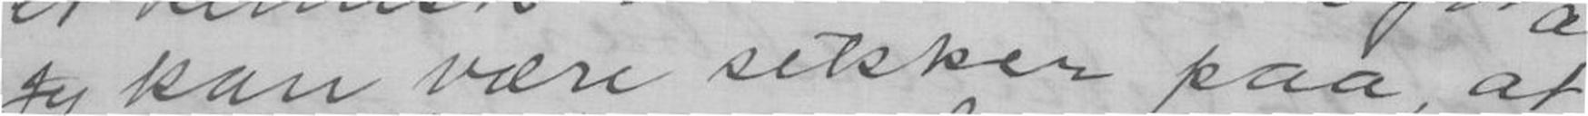jeg kan være sikker paa, at
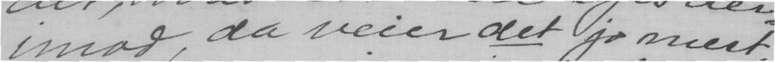imod, da veier det jo mest,
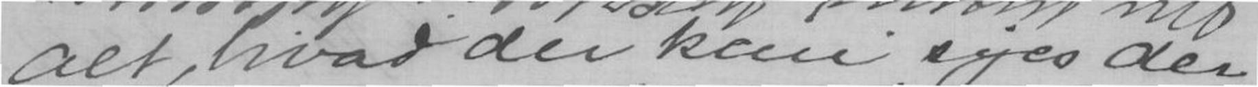Alt, hvad der kan siges der
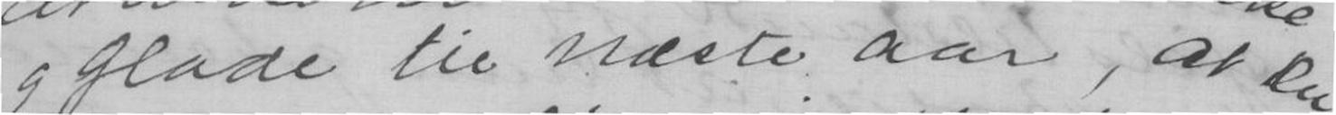og glade til næste aar, at Du
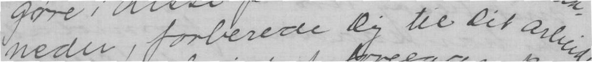neder, forberede Dig til Dit arbeide
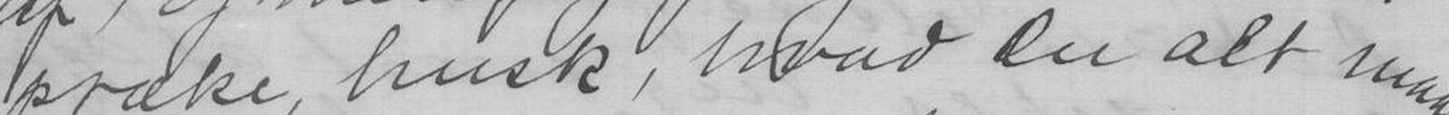præke, husk, hvad Du alt maa
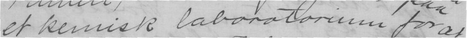et kemisk laboratorium for at
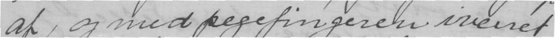af, og med pegefingeren iveiret
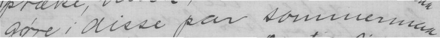göre i disse par sommermaa-
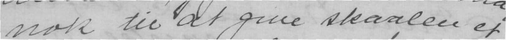nok til at give skaalen et
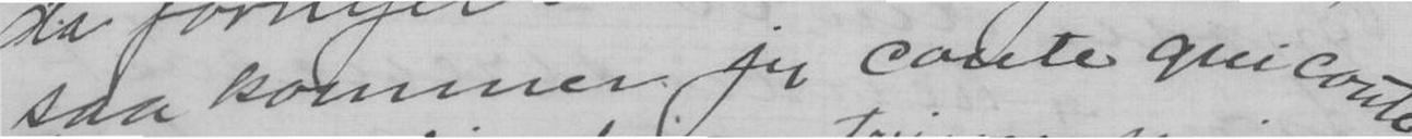saa kommer jeg coute qui coute.
